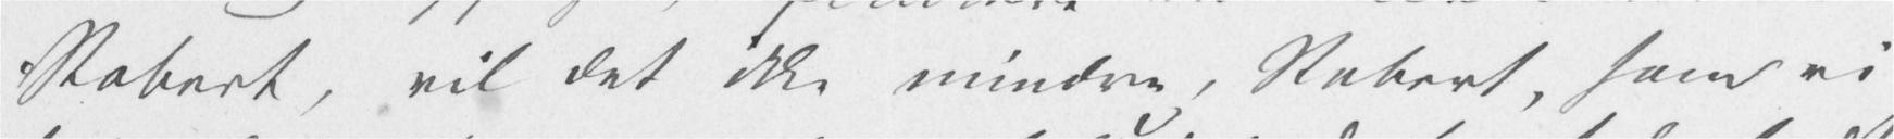Robert, vil det ikke mindre, Robert, som vi
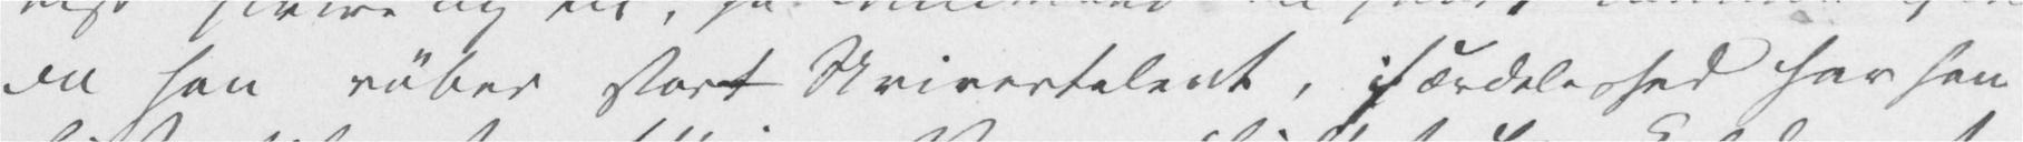da han røber stort Skrivertalent, isærdeleshed har han
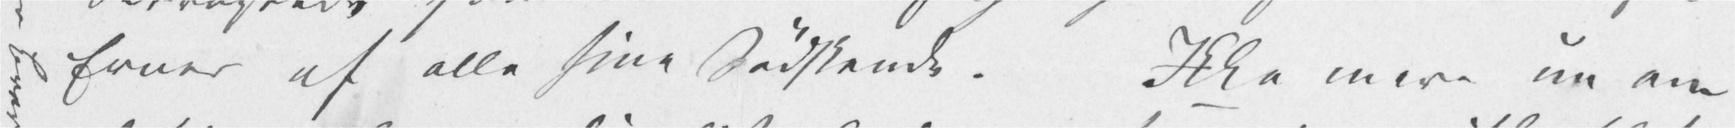Evner af alle sine Sødskende. Ikke mere nu om
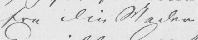fra Din Moder
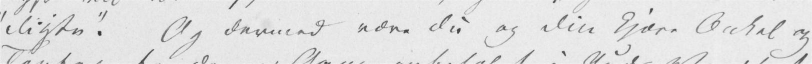«digte». Og dermed være Du og Din kjære Onkel og
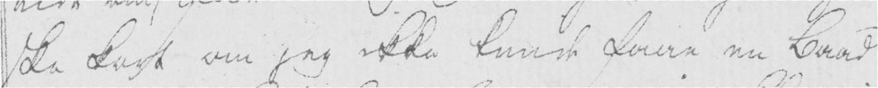ske kort om jeg ikke kunde faae en Baad
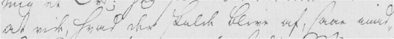at vide, hvad der skulde blive af, saae imod-
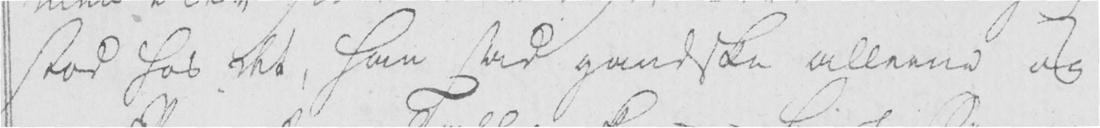stod hos det, han sad gandske alleene og
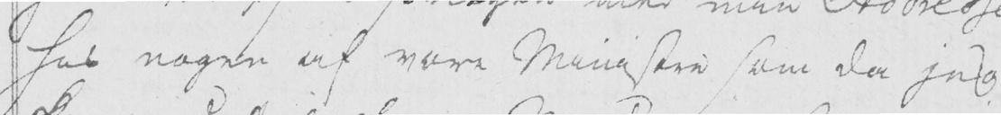hos nogen af vore Ministre som da jeg
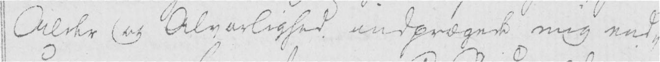Alder og Alvorlighed indprægede mig end-
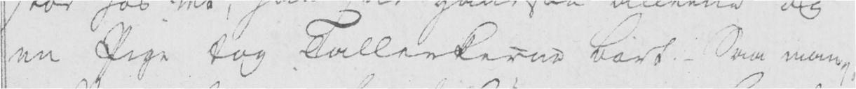en Pige tog Tallerkerne bort. - Saa man-
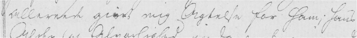allereede givet mig Agtelse for ham; hans
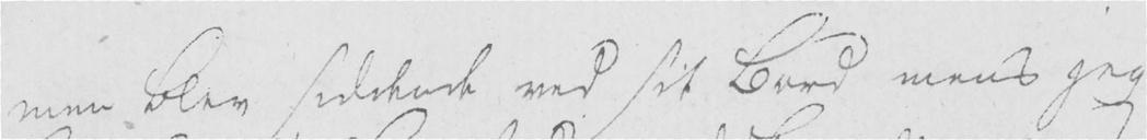men blev siddende ved sit Bord mens jeg
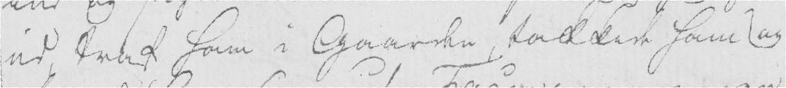ud, traf ham i Gaarden, takkede ham og
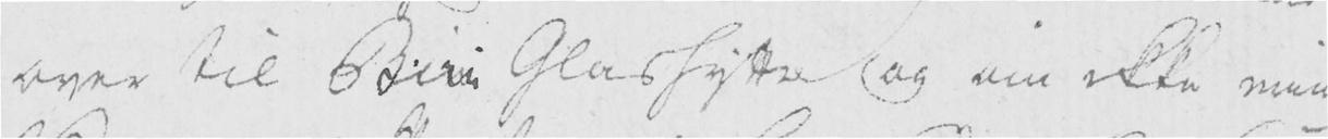over til Biri Glashytte og om ikke min
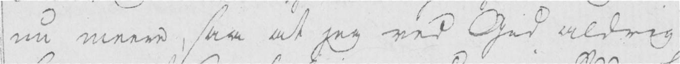nu meere, saa at jeg ved Gud aldrig
